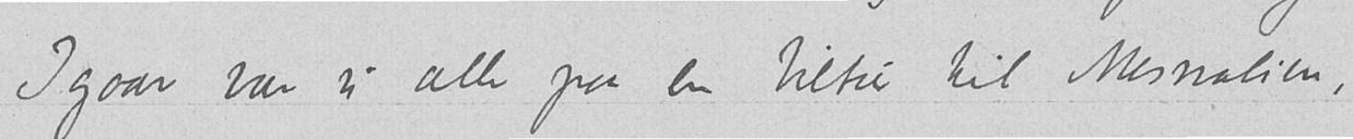Igaar var vi alle paa en biltur til Mesnalien,
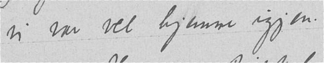vi var vel hjemme igjen.
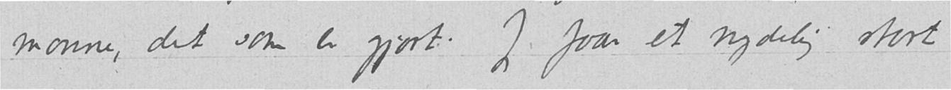monne, det som er gjort. Jeg faar et nydelig stort
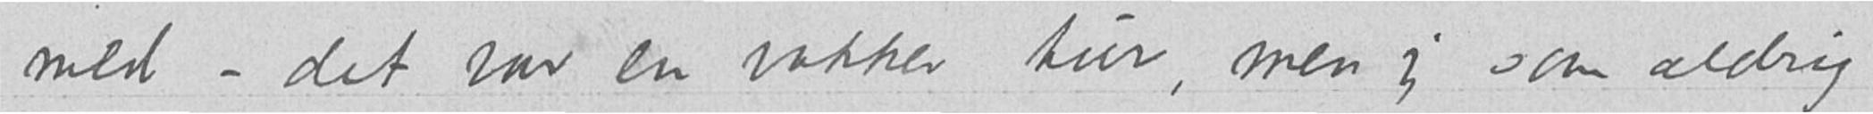med – det var en vakker tur, men jeg som aldrig
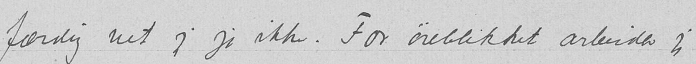færdig vet jeg jo ikke. For øieblikket arbeider jeg
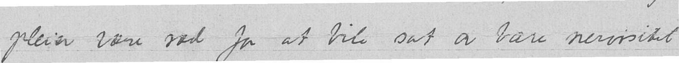pleier være ræd for at bile sat av bare nervøsitet
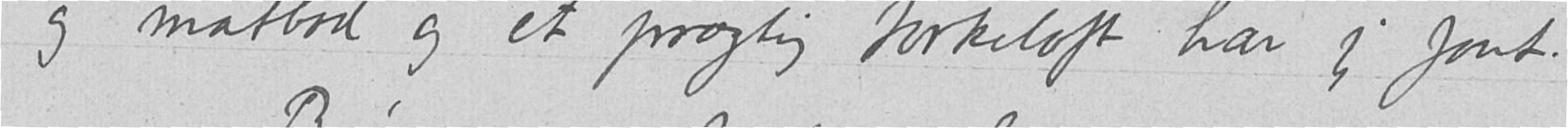og matbod og et prægtig tørkeloft har jeg faat.
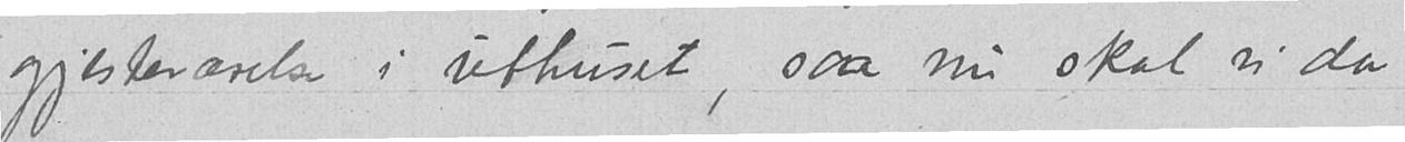gjesteværelse i uthuset, saa nu skal vi da
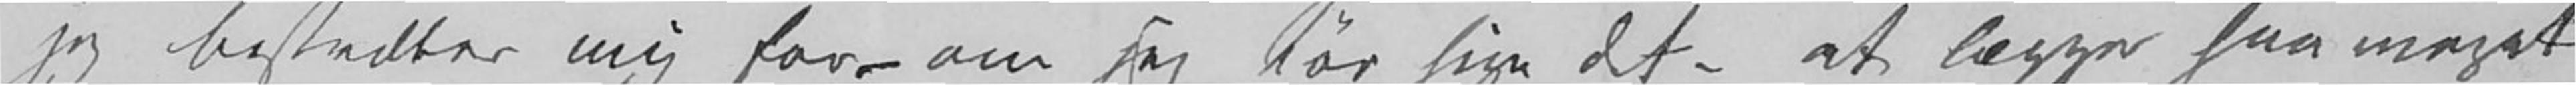jeg bestræber mig for – om jeg tør sige det – at lægge saa meget
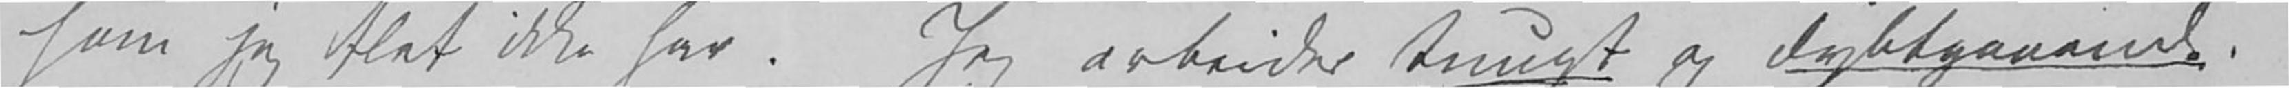som jeg slet ikke har. Jeg arbeider tungt og dybtgaaende,
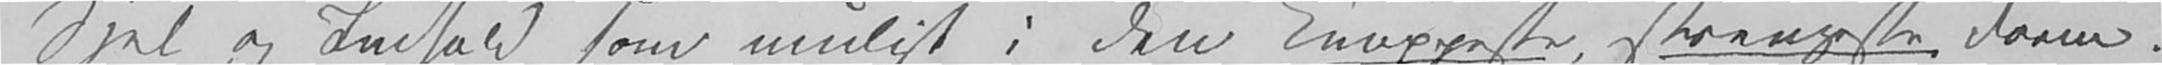Sjel og Indhold som muligt i den knappeste, strengeste Form.
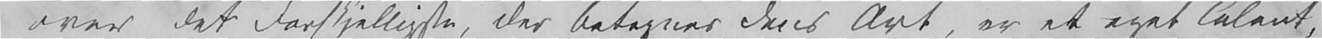over det Forskjelligste, der betegner dens Art, er et eget Talent,
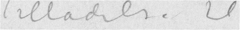Tilladelse til
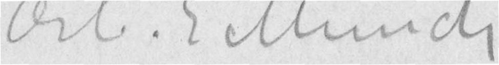Ærb. E Munch
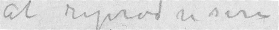at reprodusere
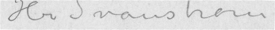Hr Svanström
